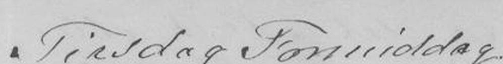Tirsdag Formiddag
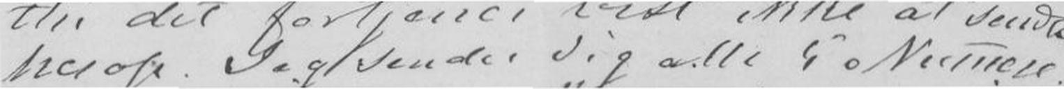herop. Jeg sender Dig alle 5 Numere,
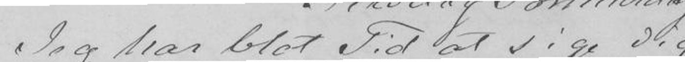Jeg har blot Tid at sige Dig
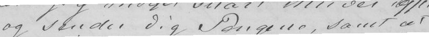og sender Dig Pengene, samt at
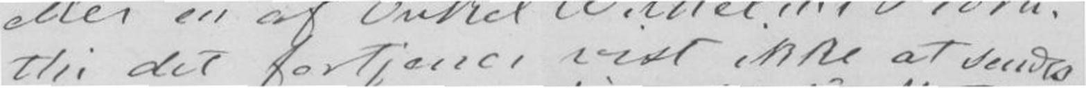thi det fortjener vist ikke at sendes
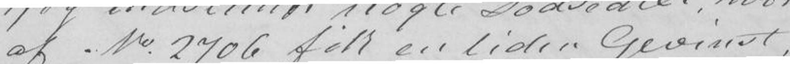af No2706 fik en liden Gevinst,
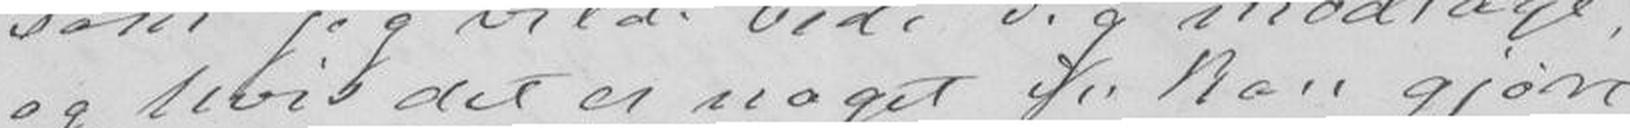og hvis det er noget Du kan gjøre
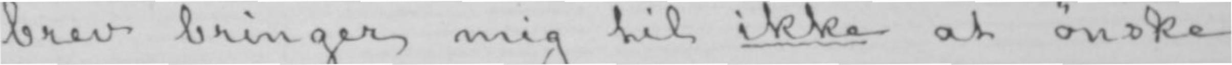brev bringer mig til ikke at ønske
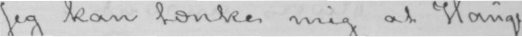Jeg kan tænke mig at Hauge-
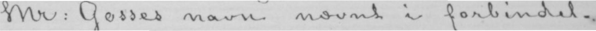Mr: Gosses navn nævnt i forbindel-
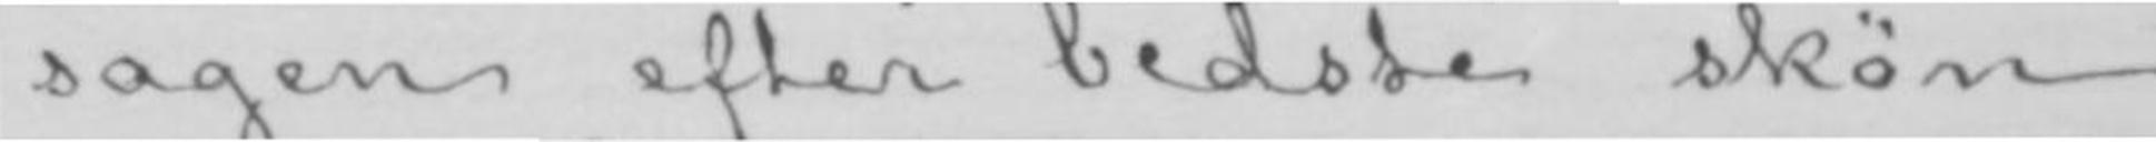sagen efter bedste skøn.-
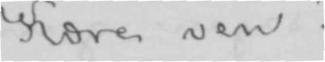Kære ven!
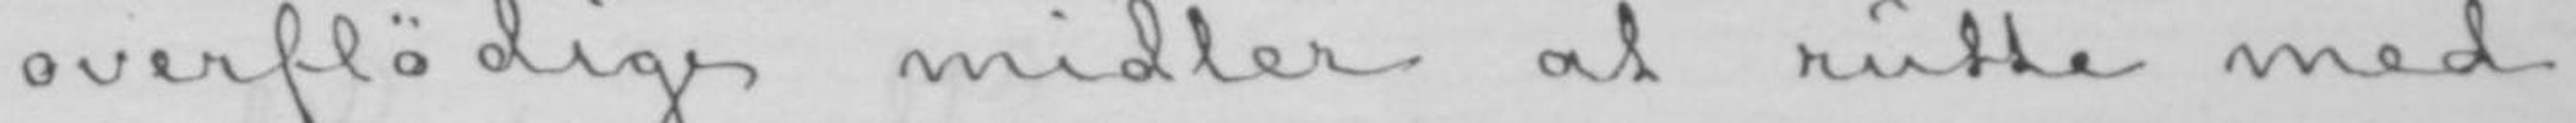overflødige midler at rutte med.
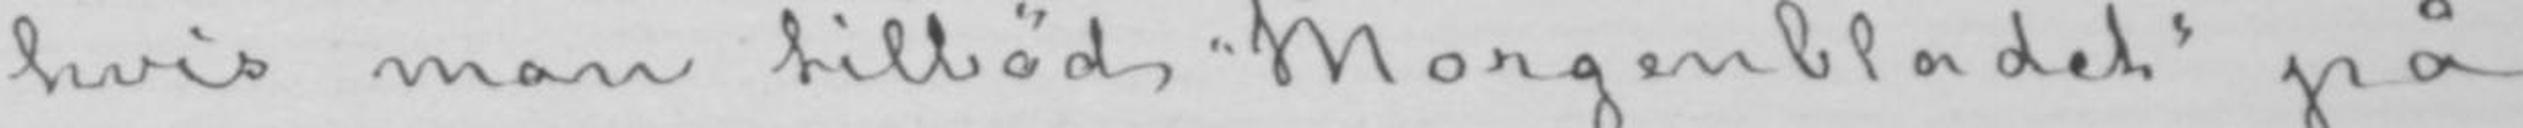hvis man tilbød «Morgenbladet» på
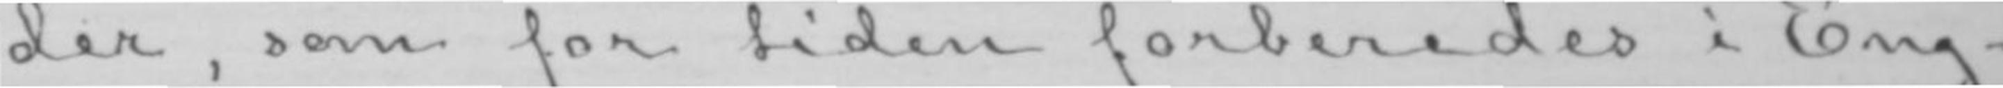der, som for tiden forberedes i Eng-
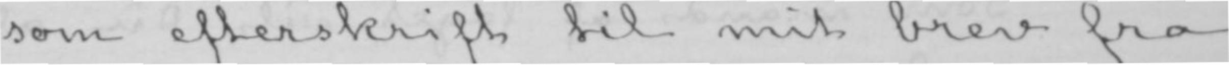som efterskrift til mit brev fra
